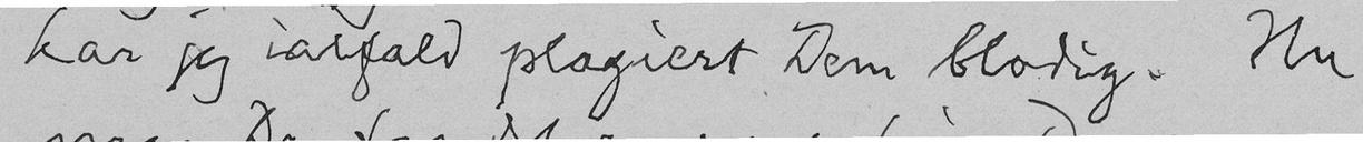har jeg ialfald plagiert Dem blodig. Nu
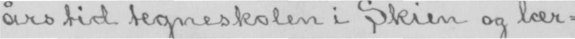års tid tegneskolen i Skien og lær-
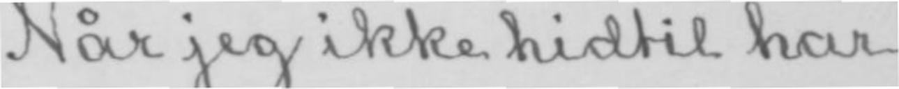Når jeg ikke hidtil har
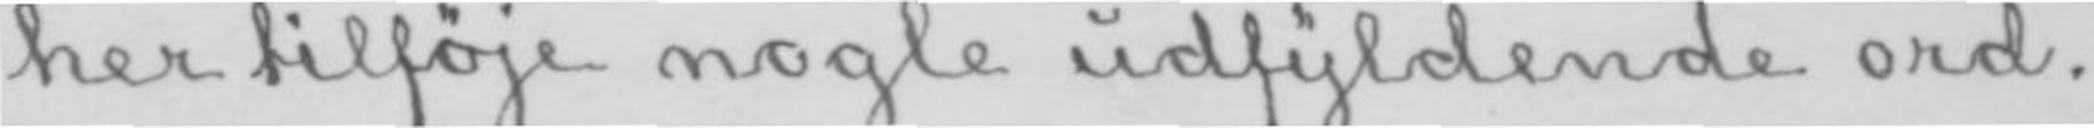her tilføje nogle udfyldende ord.
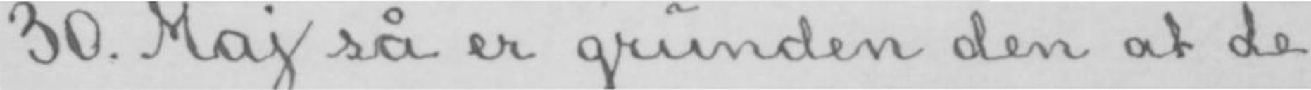30. Maj så er grunden den at de
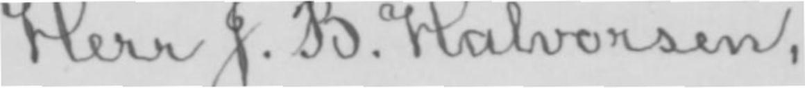Herr J. B. Halvorsen,
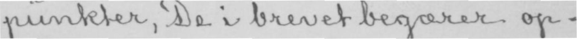punkter, De i brevet begærer op-
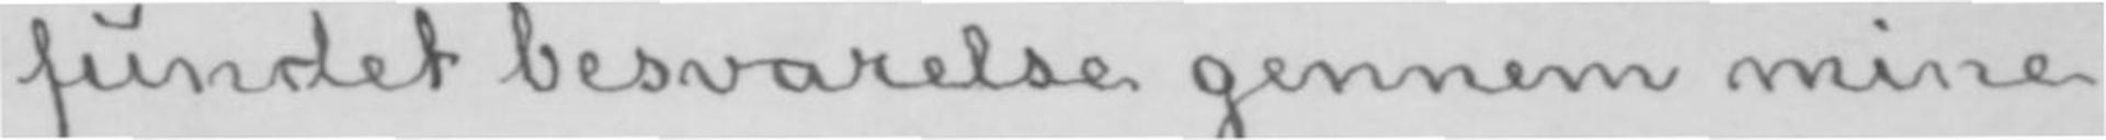fundet besvarelse gennem mine
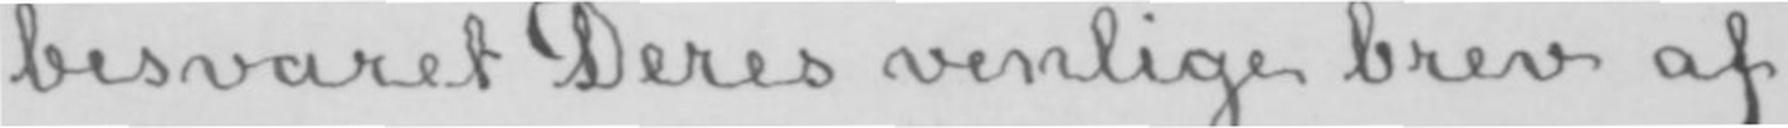besvaret Deres venlige brev af
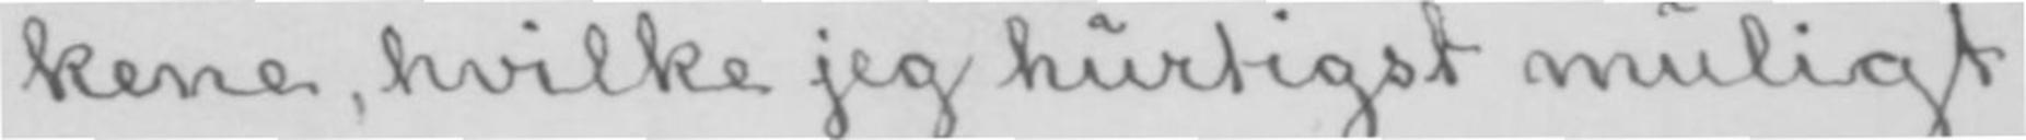kene, hvilke jeg hurtigst muligt
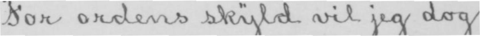For ordens skyld vil jeg dog
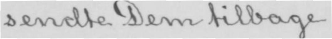sendte Dem tilbage.
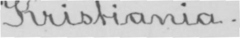Kristiania.
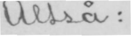Altså:
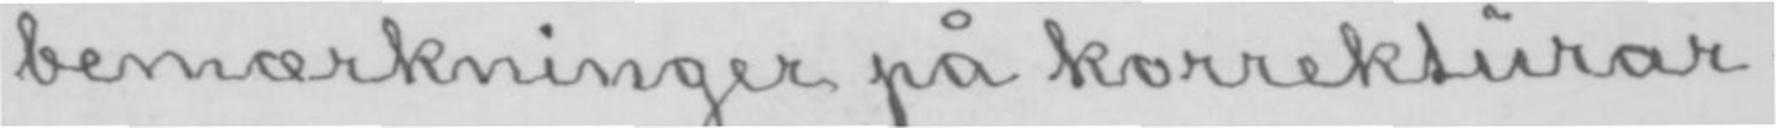bemærkninger på korrekturar-
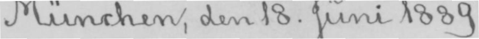München, den 18. Juni 1889.
